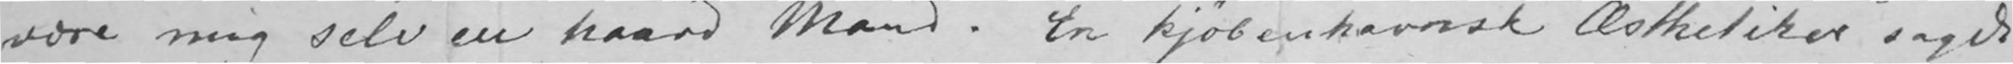være mig selv en haard Mand. En kjøbenhavnsk Æsthetiker sagde
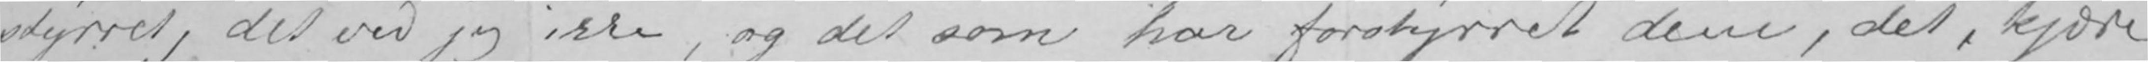styrret, det ved jeg ikke, og det som har forstyrret dem, det, kjære
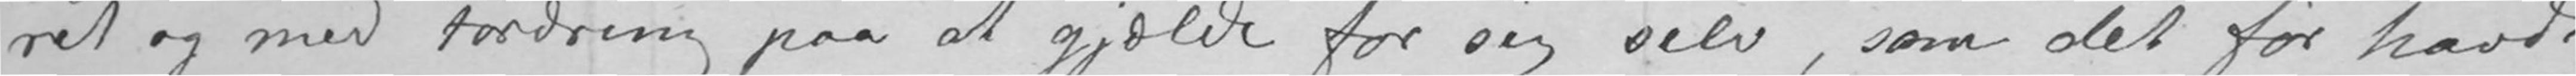ret og med Fordring paa at gjælde for sig selv, som det før havde
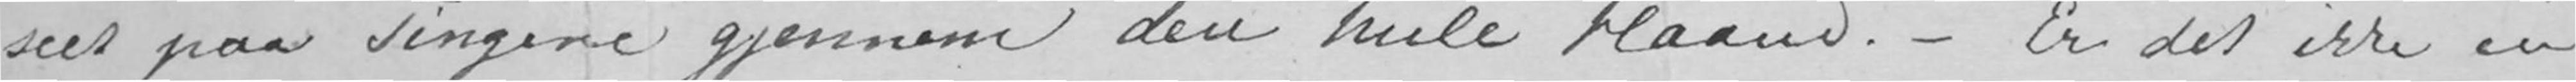seet paa Tingene gjennem den hule Haand.- Er det ikke en
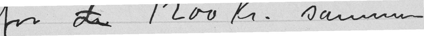for d 1200 Kr. sammen
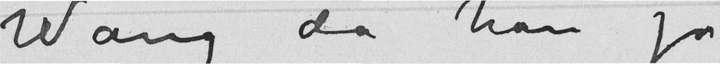Wang da han jo
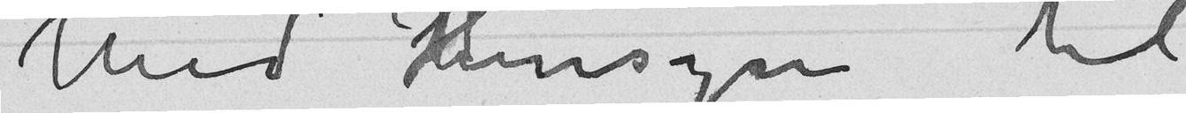Med Hensyn til
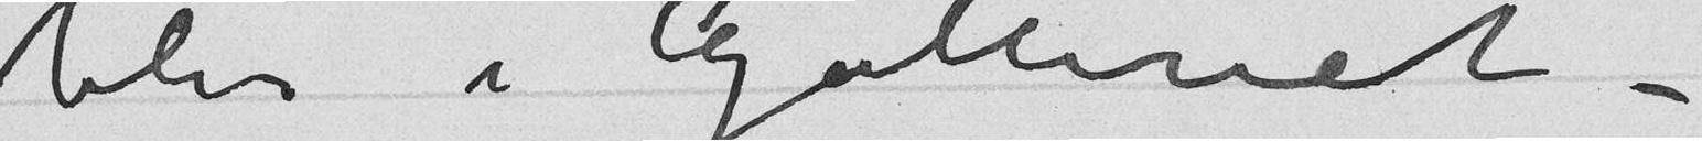blir i Galleriet –
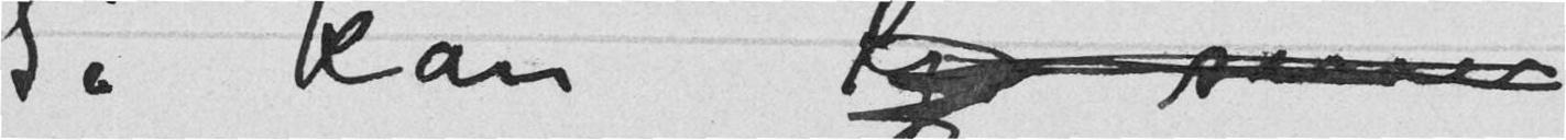Så kan Kj
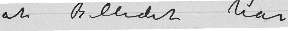at Billedet har
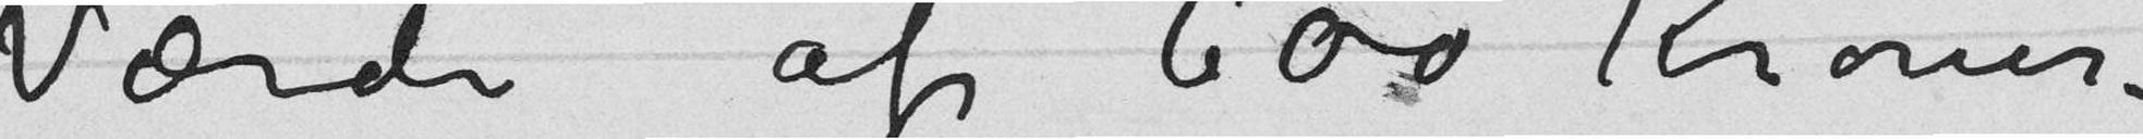Værdi af 600 Kroner –
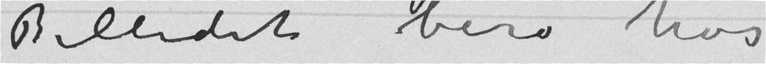Billedet bero hos
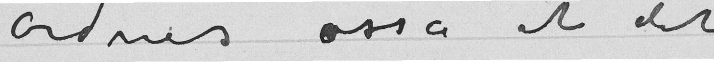ordnes osså at det
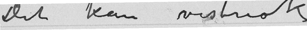Det kan vistnok
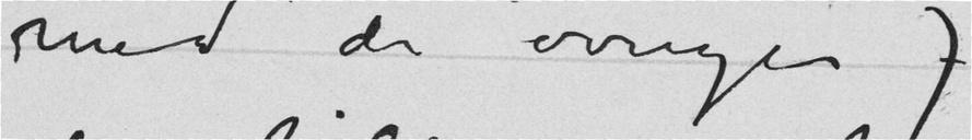med de øvrige)
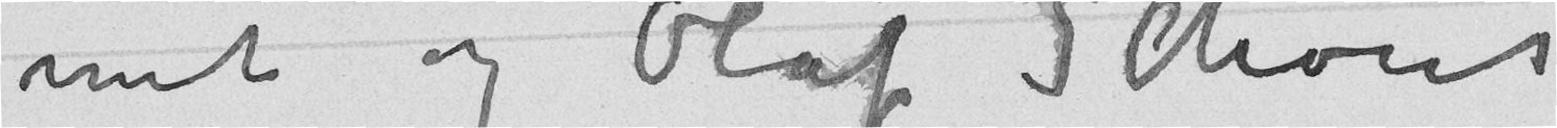mit og Olaf Schous
